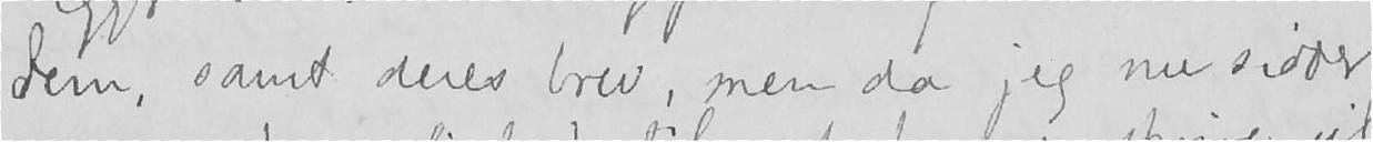dem, samt deres brev, men da jeg nu sidder
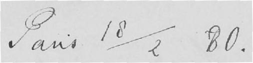Paris 18/2 80.
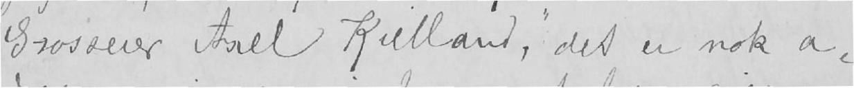«Grosserer Axel Kielland», det er nok a-
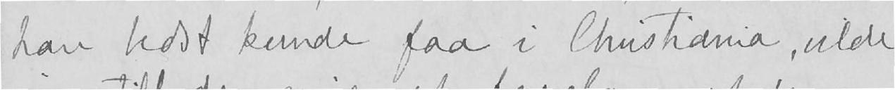han bedst kunde faa i Christiania, vilde
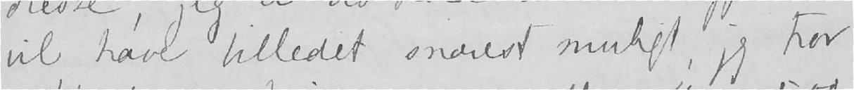vil have billedet snarest muligt, jeg tror
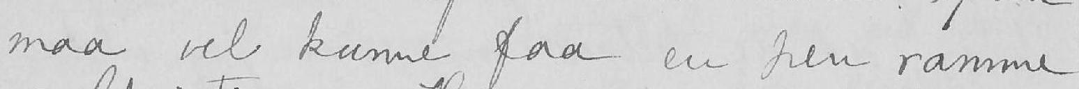maa vel kunne faa en pen ramme
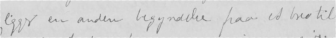ligger en anden begyndelse paa et brev til
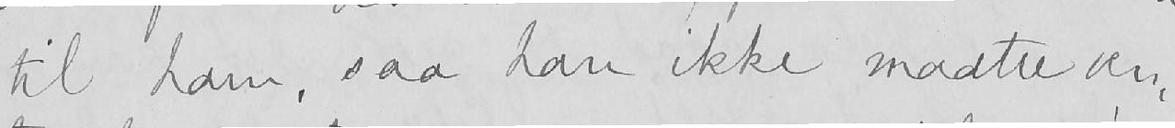til ham, saa han ikke maatte ven-
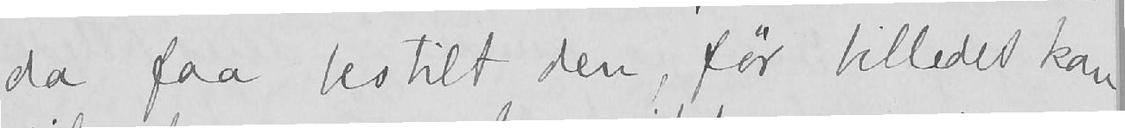da faa bestilt den, før billedet kom
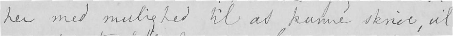her med mulighed til at kunne skrive, vil
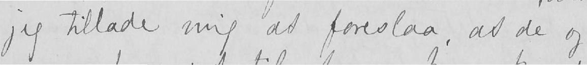jeg tillade mig at foreslaa, at de og-
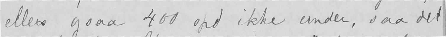ellers ogsaa 400 spd ikke under, saa det
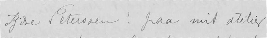Kjære Peterssen! paa mit atélier
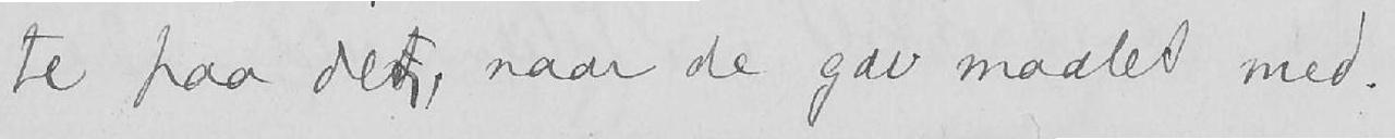te paa det naar de gav maalet med.
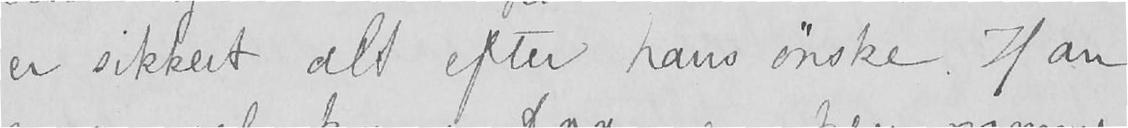er sikkert alt efter hans ønske. Han
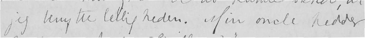jeg benytte leiligheden. Min oncle hedder
